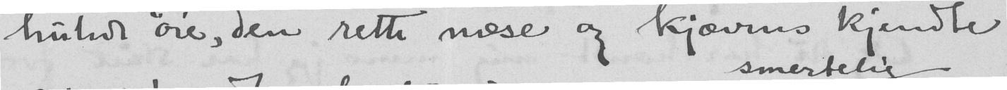hulede øie, den rette næse og kjævens kjendte
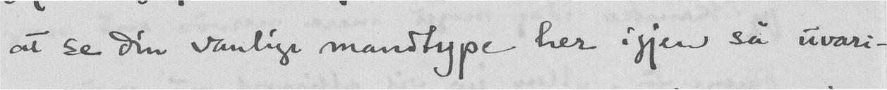at se din vanlige mandtype her igjen så uvari-
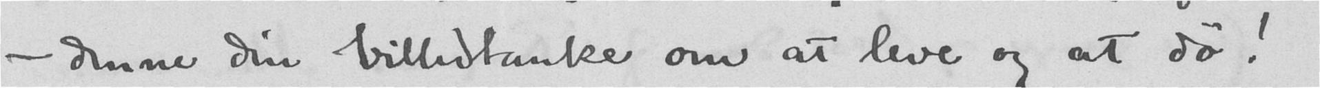- denne din billedtanke om at leve og at dø!
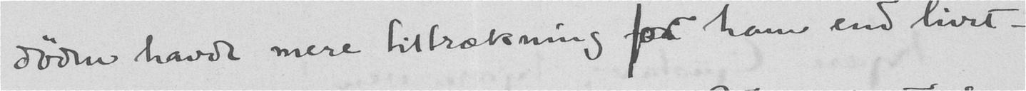døden havde mere tiltrækning for ham end livet -
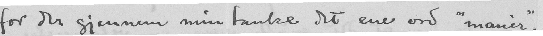for der gjennem min tanke det ene ord, "manèr".
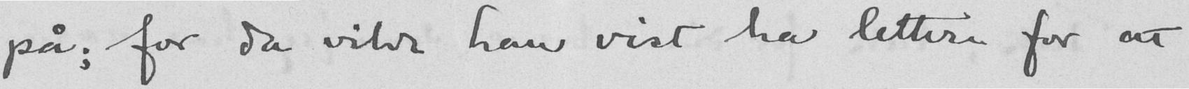på; for da vilde han vist ha lettere for at
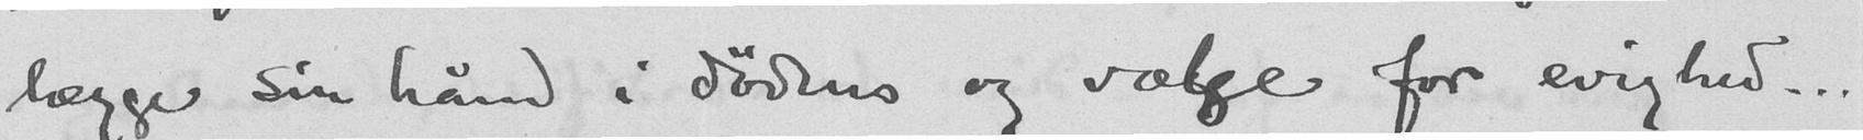lægge sin hånd i dødens og vælge for evighed..
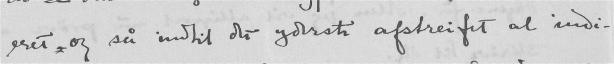eret, og så indtil det yderste afstreifet al indi-
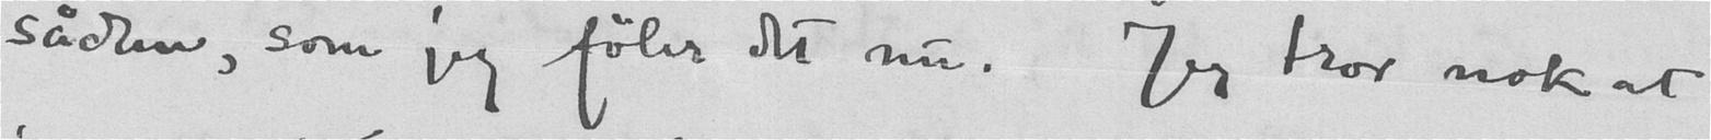sådan, som jeg føler det nu. Jeg tror nok at
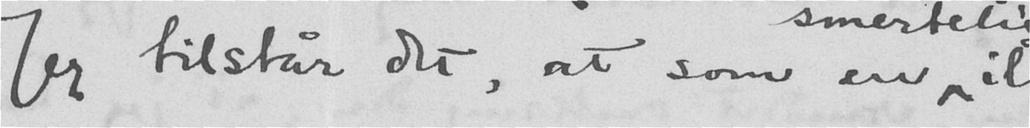Jeg tilstår det, at som en
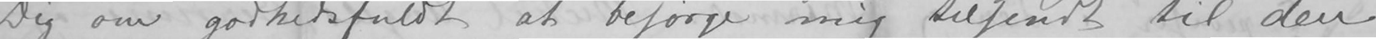Dig om godhedsfuldt at besørge mig tilsendt til den
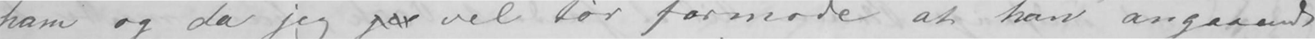ham og da jeg je vel tør formode at han angaaende
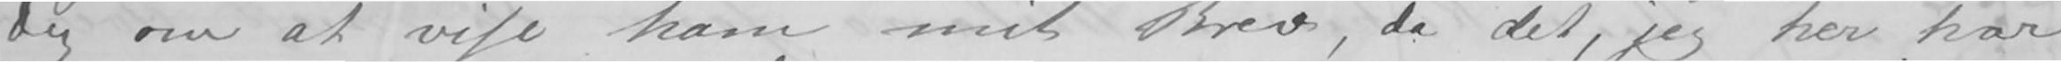Dig om at vise ham mit Brev, da det, jeg her har
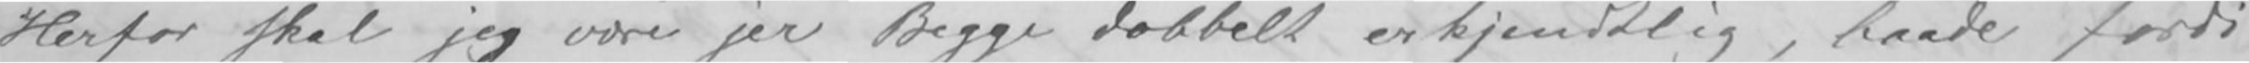Herfor skal jeg være jer Begge dobbelt erkjendtlig, baade fordi
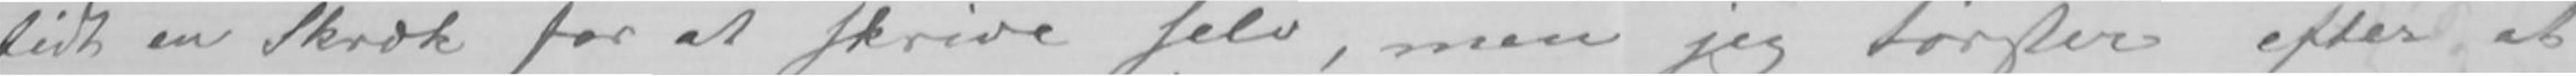tidt en Skræk for at skrive selv, men jeg tørster efter at
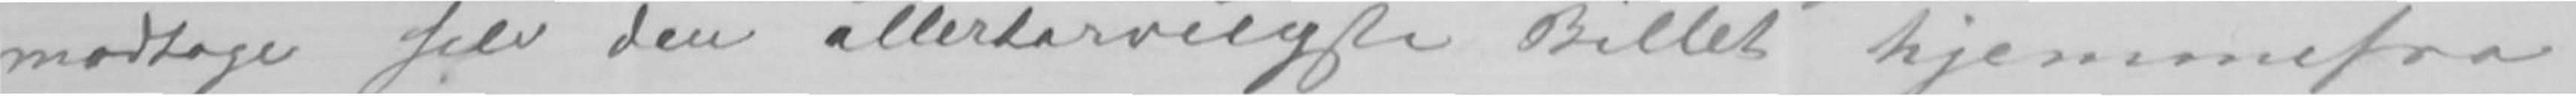modtage selv den allertarveligste Billet hjemmefra.
